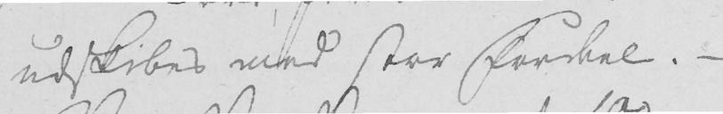udskibes med stor Fordel. -
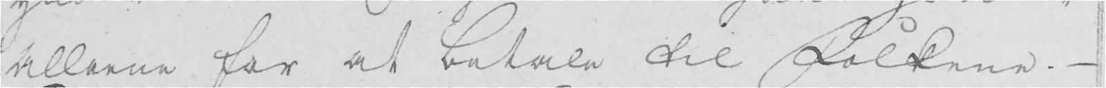alleene for at betale til Folkene. -
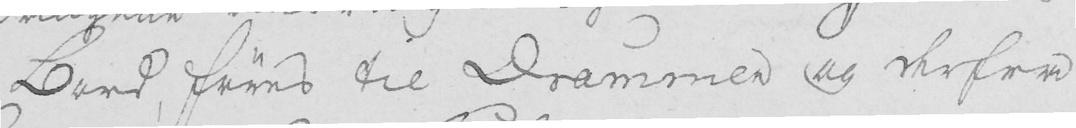Bord, føres til Drammen og derfra
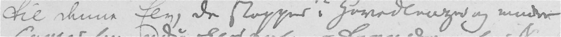til denne Elv, de stopper i Hovedlenze og under
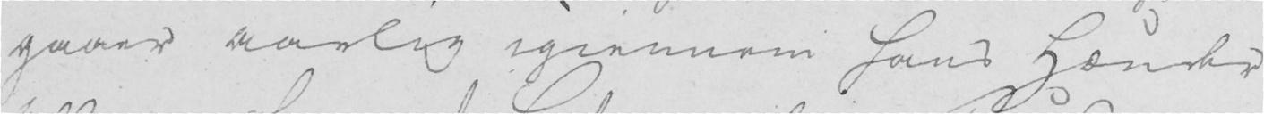gaaer aarlig igiennem hans Hænder
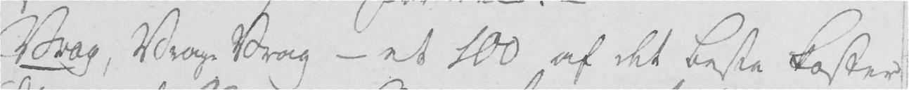Vrag, Vrage Vrag - et 100 af det beste koster
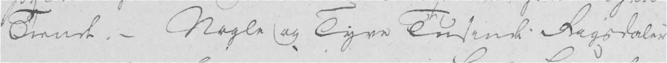Tiende. Nogle og Tyve Tusinde Rigsdaler
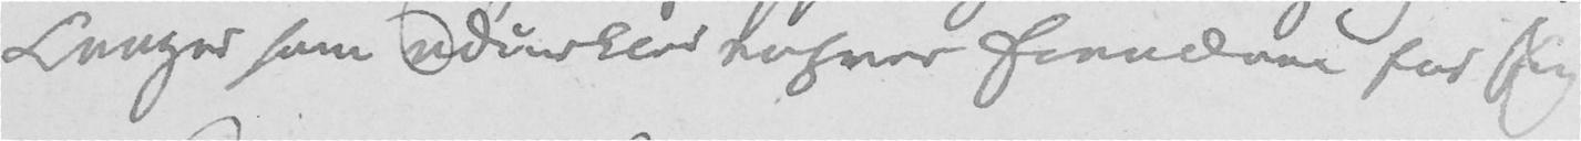Lenzer som udvirker enhver foruden for slig
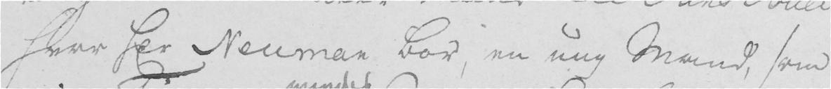hvor Hr Neuman bor, en ung Mand, som
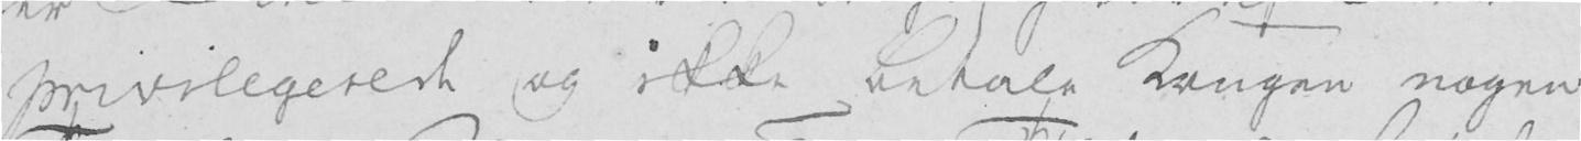privilegerede og ikke betale Kongen nogen
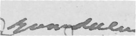ramdalen
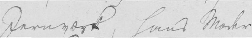Jernværk, hans Moder
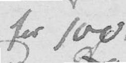for 100
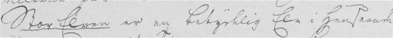Stor Elven er en betydelig Elv i henseende
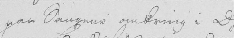paa Saugene omkring i D
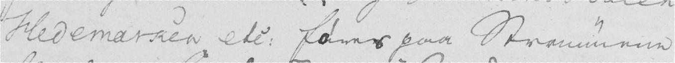Hedemarken etc. føres paa Strømmene
